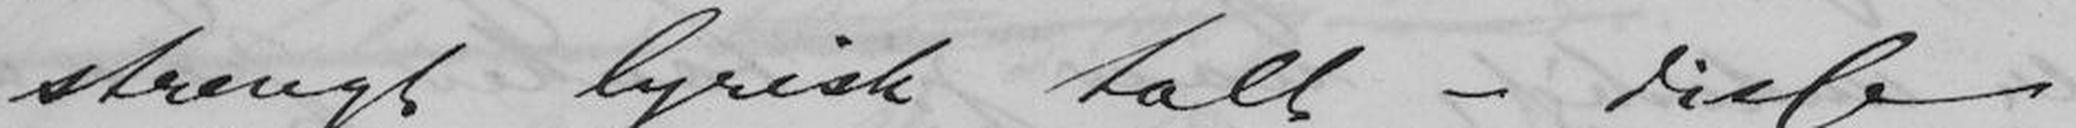strengt lyrisk talt - disse
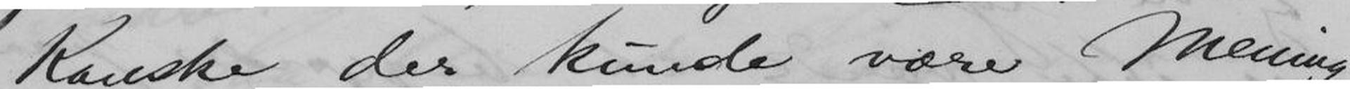Kanske der kunde være Mening
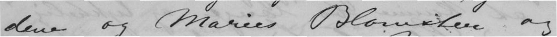dene og Maries Blomster og
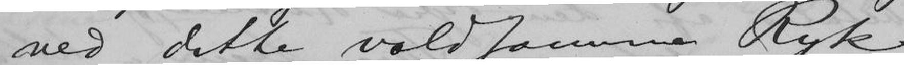ved dette voldsomme Ryk
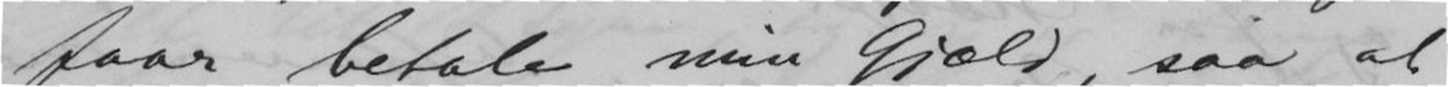faar betale min Gjæld, saa at
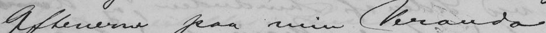Aftenerne paa min Veranda
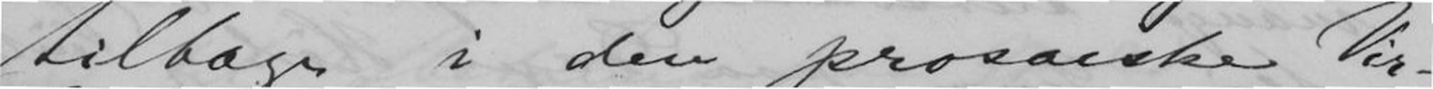tilbage i den prosaiske Vir-
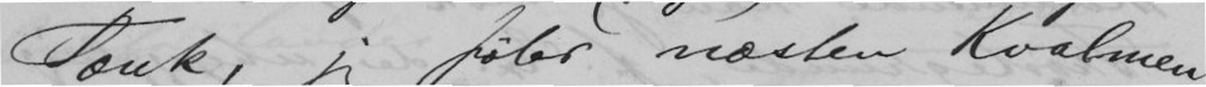Tænk, jeg føler næsten Kvalmen
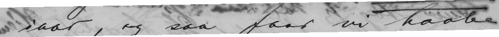iaar, og saa faar vi haabe
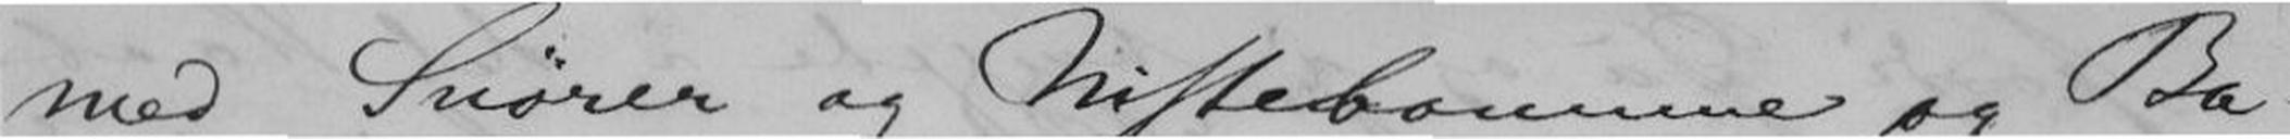med Snører og Nistebomme og Ba-
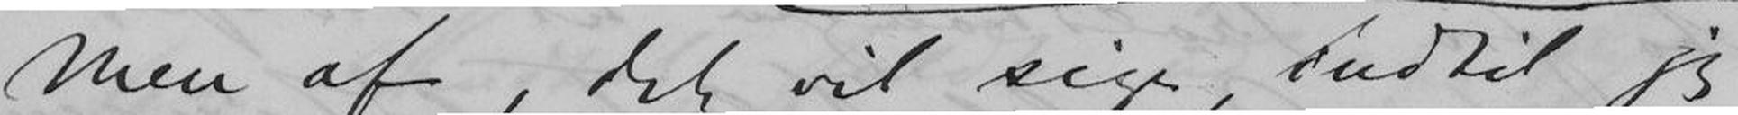men af, det vil sige, indtil jeg
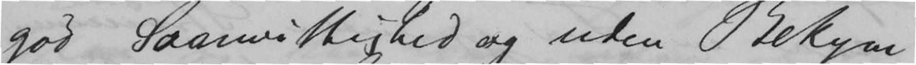god Samvittighed og uden Bekym-
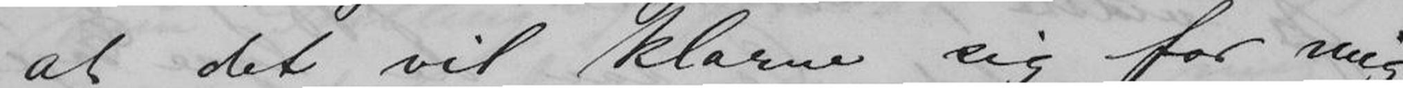at det vil klare sig for mig
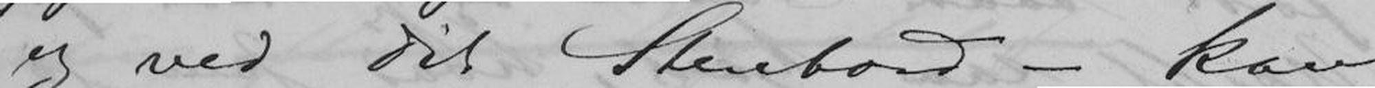og ved dit Stenbord - kan
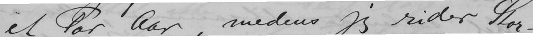et Par Aar, medens jeg rider Stor-
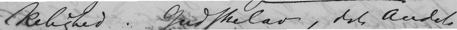kelighed. Gudskjelov, det Andet
